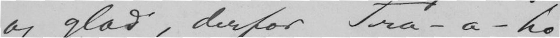og glad, derfor Tra-a-ho,
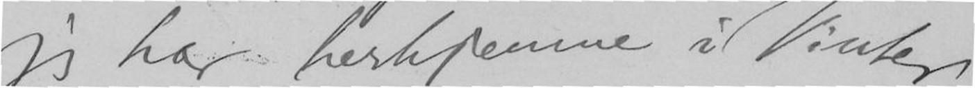jeg har herhjemme i Vinter.
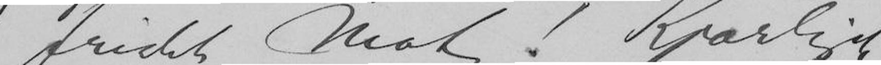og fridst Mot! Kjærligste
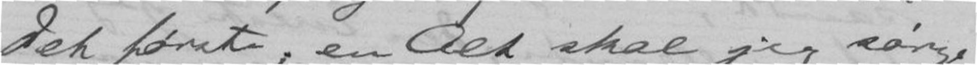det første; en Alt skal jeg sørge
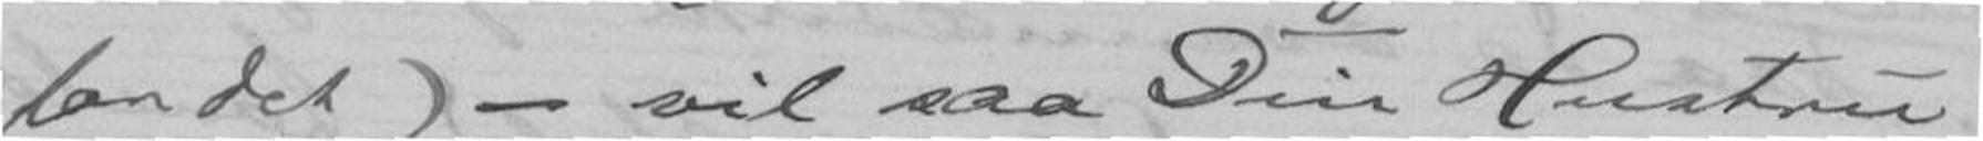for det)- vil saa Din Hustru
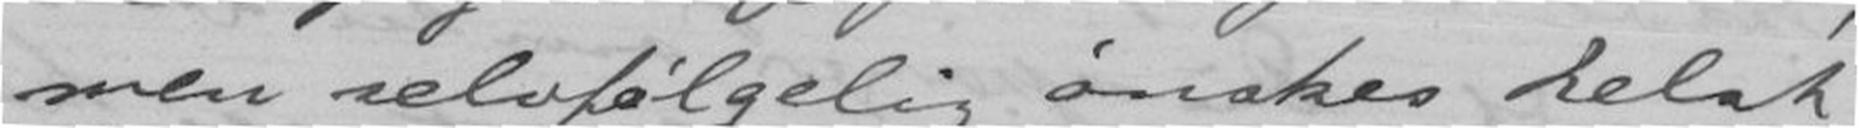men selvfølgelig ønskes helst
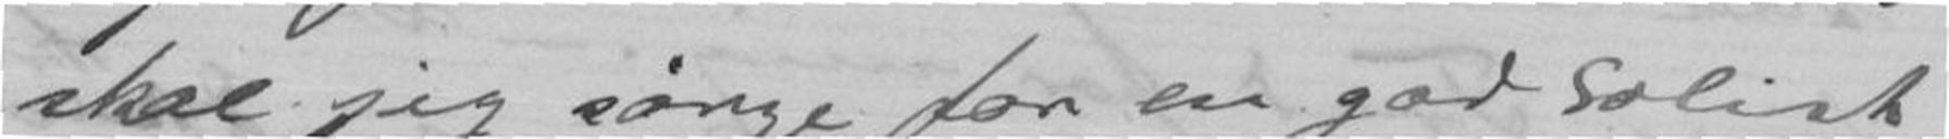skal jeg sørge for en god solist,
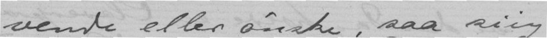vende eller ønske, saa siig
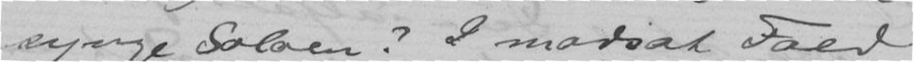synge Soloen? I modsat Fald
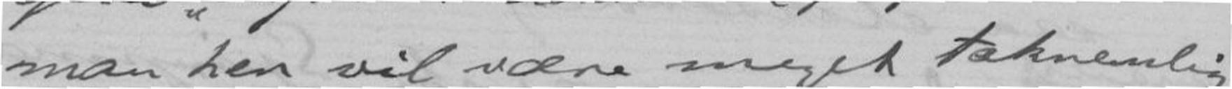man her vil være meget taknemlig
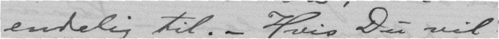endelig til.- Hvis Du vil
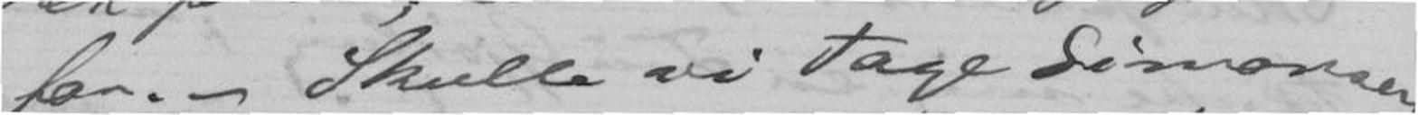for. Skulle vi tage Simonsen
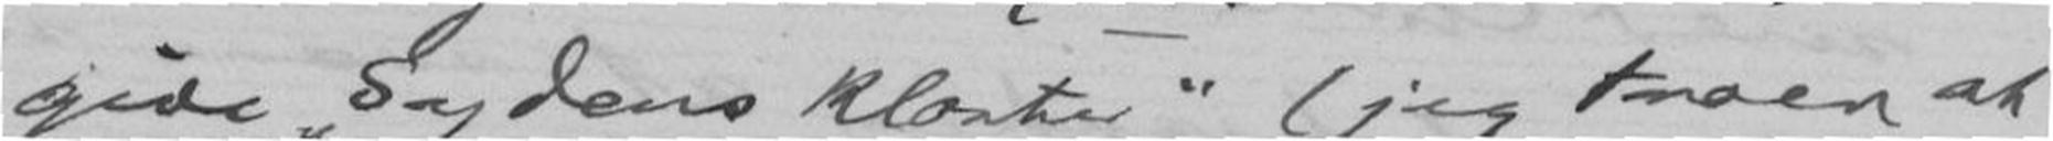give "Sydens Kloster" (jeg troer at
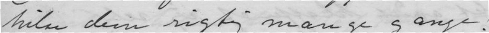hilse dem rigtig mange gange!
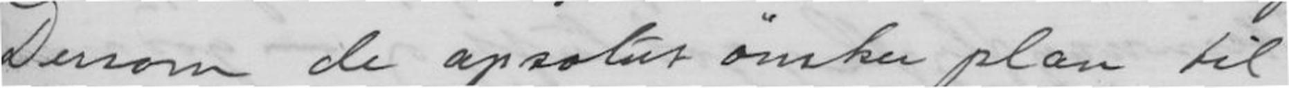Dersom de apsolut ønsker plan til
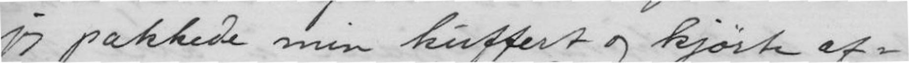jeg pakkede min kuffert og kjørte af-
